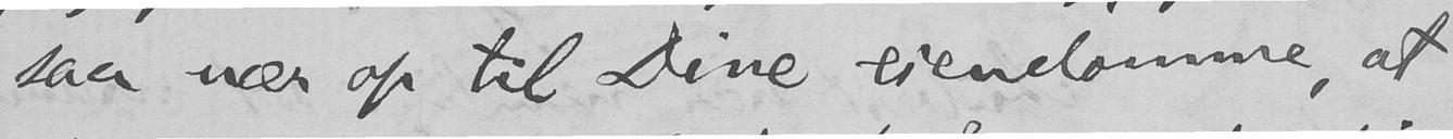saa nær op til Dine eiendomme, at
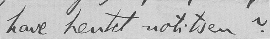have hentet notitsen?
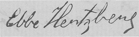Ebbe Hertzberg.
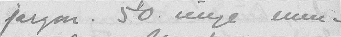jargon. 50 unge men-
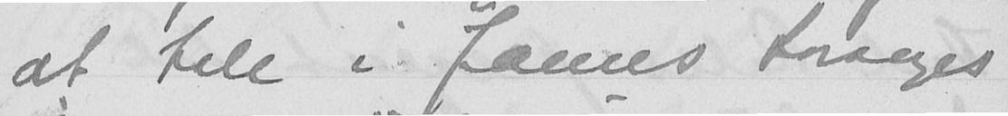at tale i James Loranges
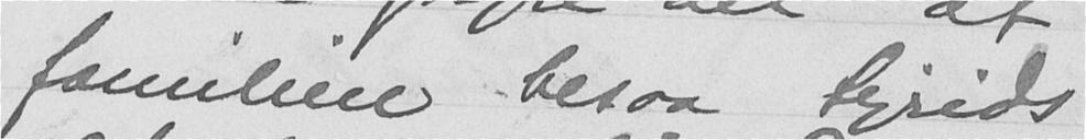familien besaa Sigrids
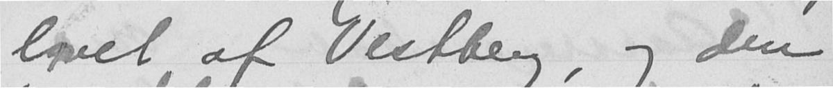lavet af Vestberg, og den
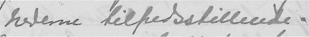hederne tilfredsstillende.
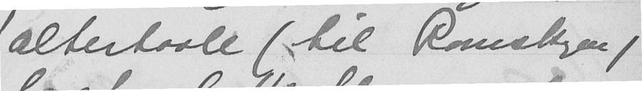altertavle (til Romskogen)
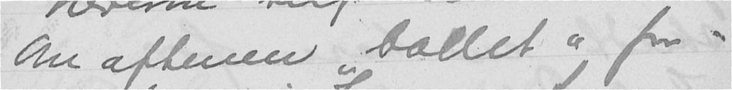Om aftenen "ballet" for
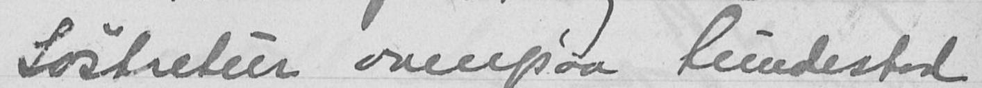Søstretur ovenpaa Lundestad
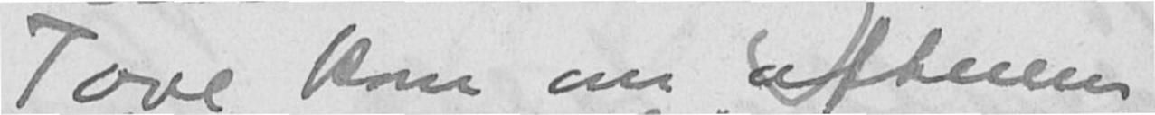Tove kom om aftenen
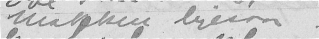Makken ligesaa.
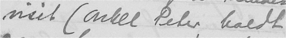visit (Onkel Peter holdt
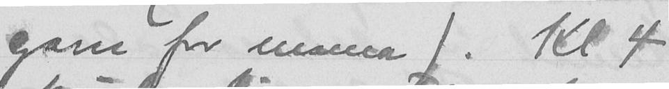garn for mama). Kl 4
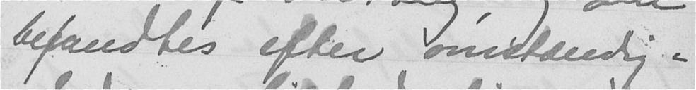befandtes efter omstændig-
